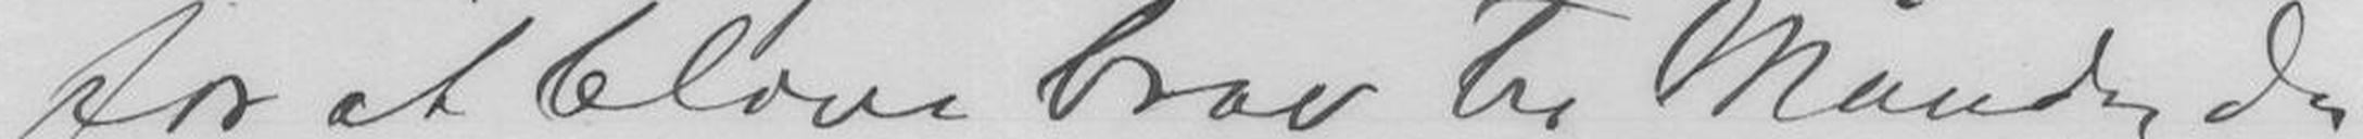for at blive brad til Mandag da
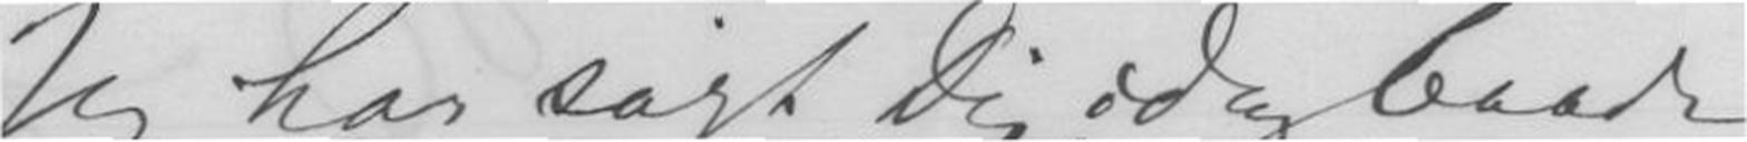Jeg har sagt Dig idag baade
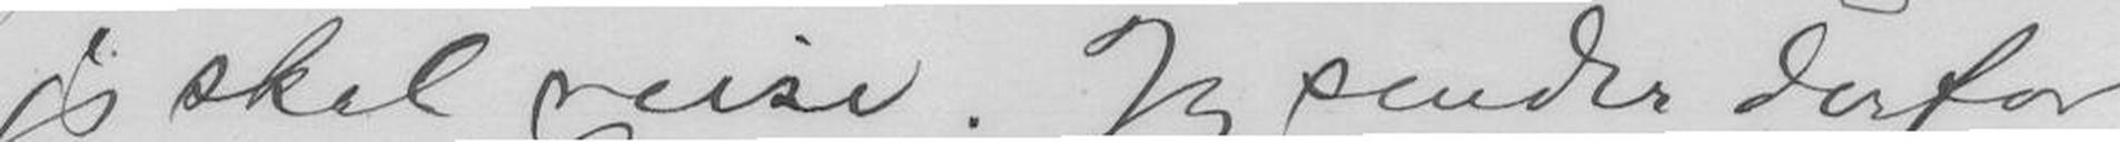jeg skal reise. Jeg sender derfor
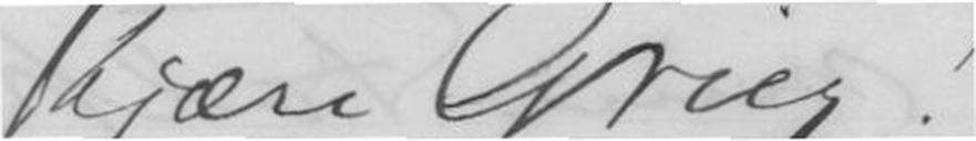Kjære Grieg!
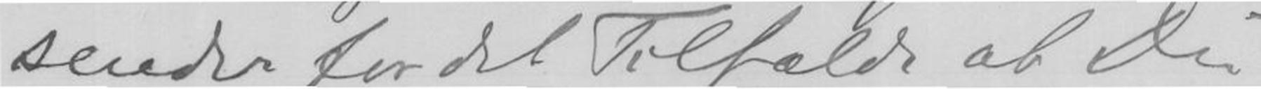sender for det Tilfælde at Du
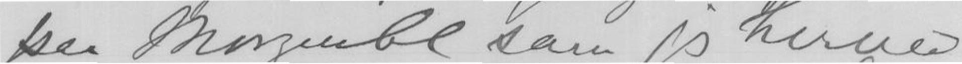paa Morgenbl som jeg herved
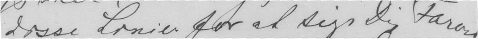disse Linier for at sige Dig Farvel
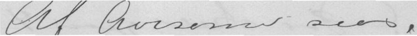Af Aviserne sees,
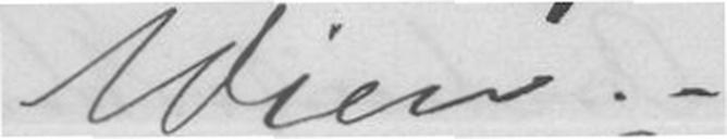Wien. -
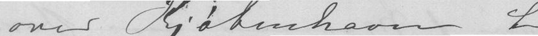over Kjøbenhavn til
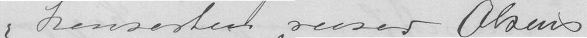konserten reiser Olsens
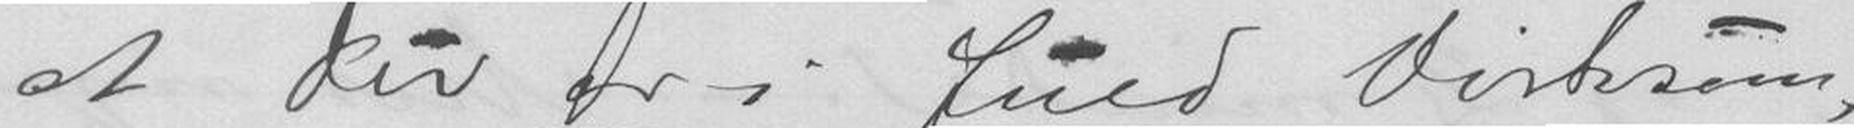at Du er i fuld Virksom
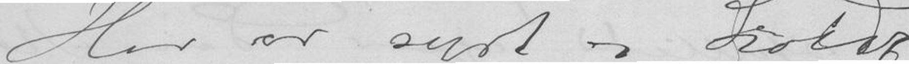Her er surt og koldt
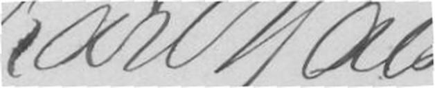Karl Hals
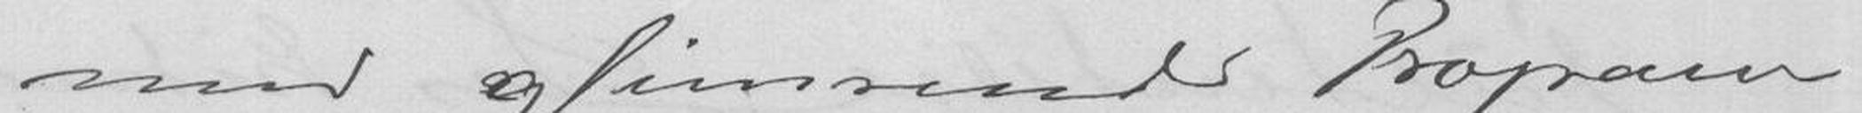med glimrende Program
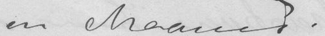en Maaned.
